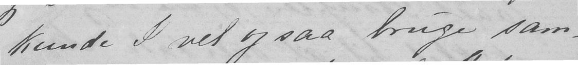kunde I vel ogsaa bruge sam-
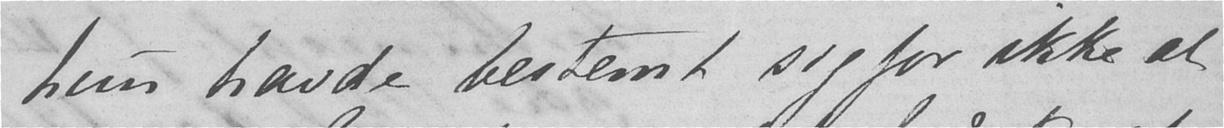hun havde bestemt sig for ikke at
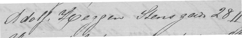Adolf Heggen Stensgade 28 II
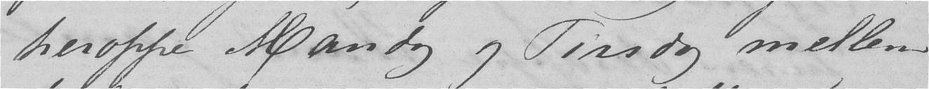heroppe Mandag og Tirsdag mellem
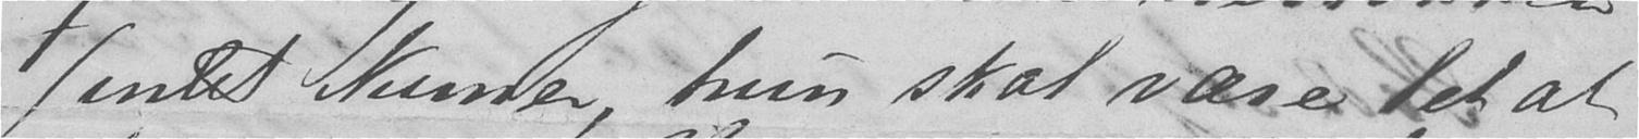(intet Numer, hun skal være let at
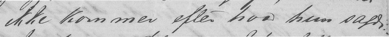ikke kommer efter hvad hun sagde.
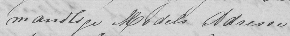mandlige Models Adresse
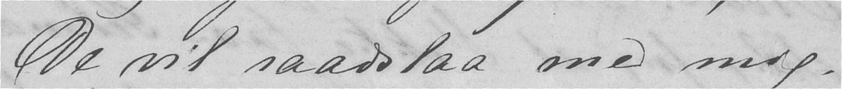De vil raadslaa med mig.
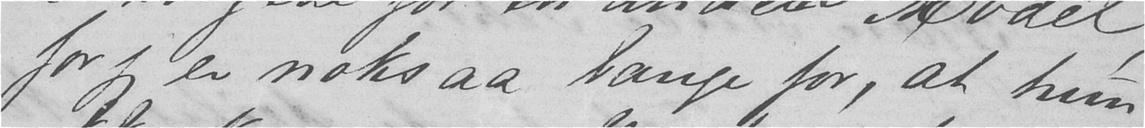for jeg er noksaa bange for, at hun
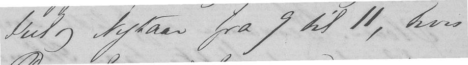Jul og Nytaar fra 9 til 11, hvis
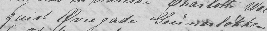quist Øvre gade Grünerløkken
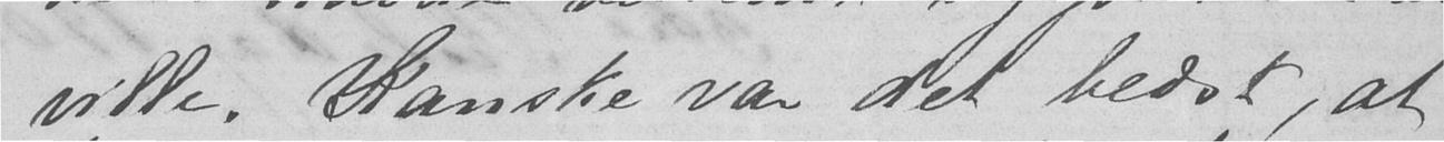ville. Kanske var det bedst, at
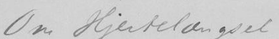Om Hjertelængsel
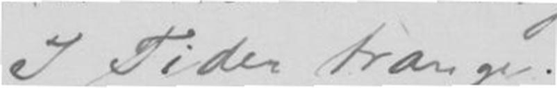I Tider Trange;
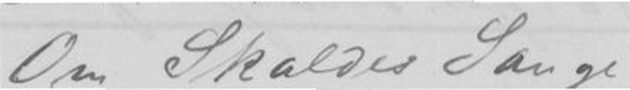Om Skalder Sange
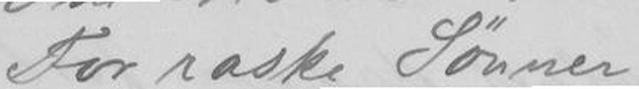For raske Sønner
